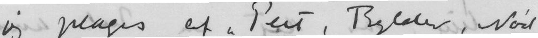jeg plages af Pest, Bylder, Nød
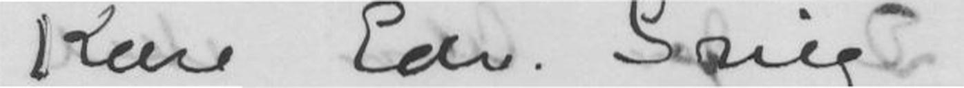Kære Edv. Grieg!
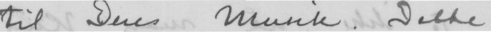til Deres Musik. Dette
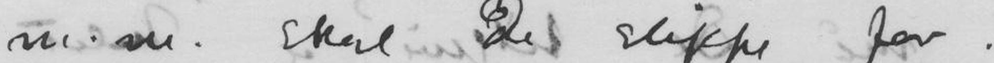m. m. skal De slippe for.
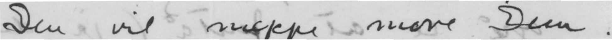Den vil næppe more Dem.
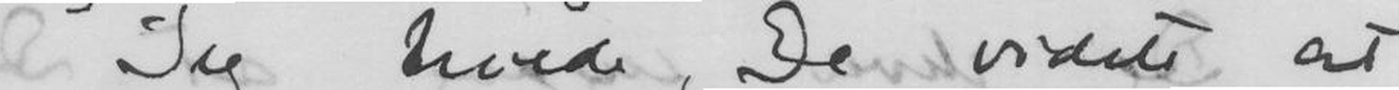Jeg troede De videst at
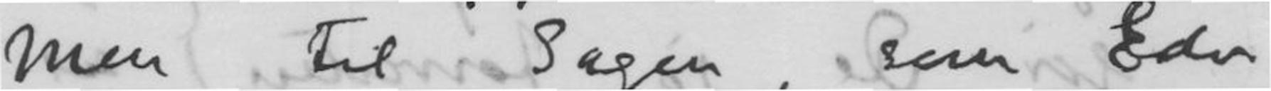Men til Sagen, som Edv
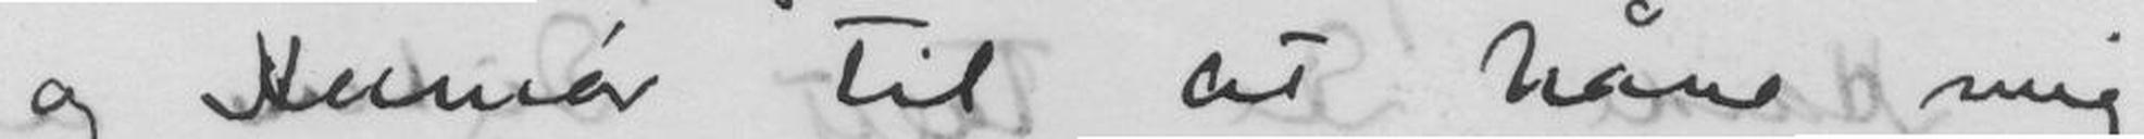og Humør til at håne mig
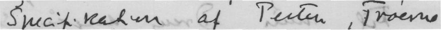Specifikation af Pesten, Trøerne
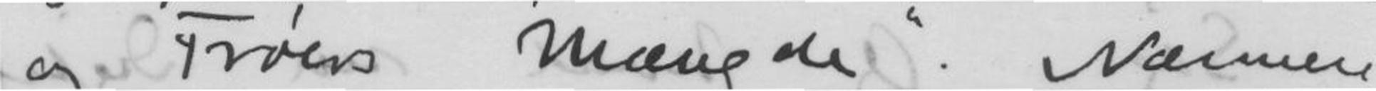og Trøers Mængde. Nærmere
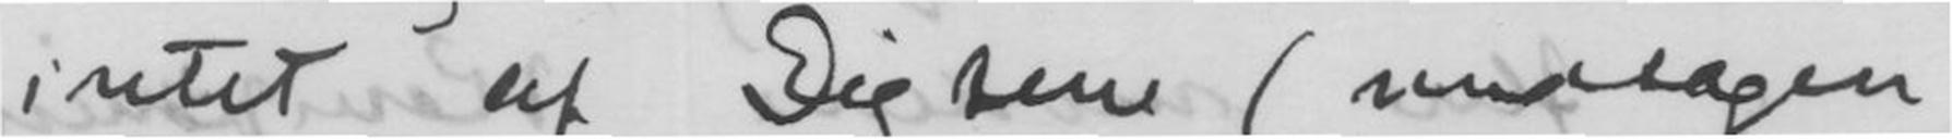intet af Digtene (undtagen
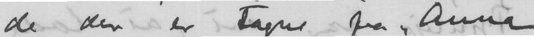de der er tagne fra Anna
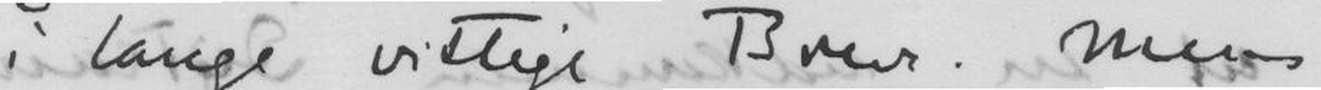i lange vittige Breve. Mens
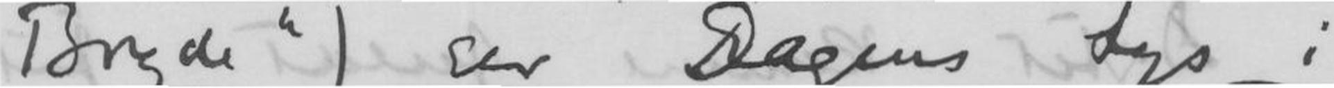Bryde) ser Dagens Lys i
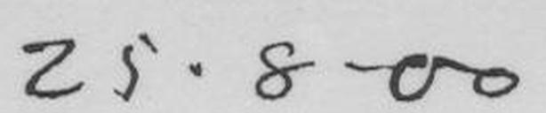25.8.00
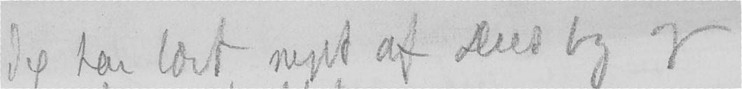Jeg har lært meget af Deres bog og
